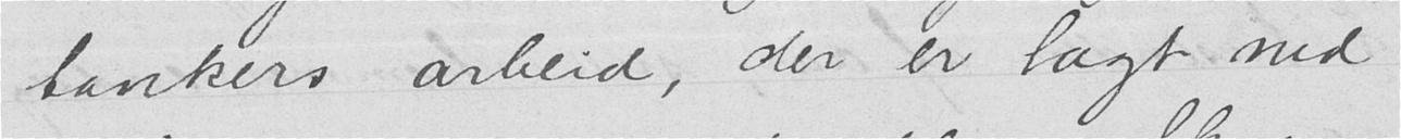tankers arbeid, der er lagt ned
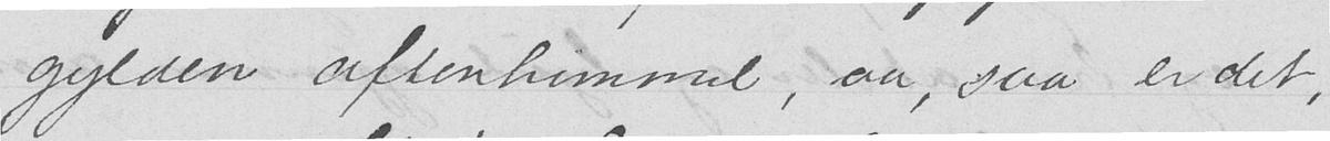gylden aftenhimmel, aa, saa er det,
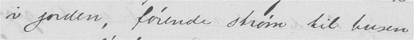i jorden, førende strøm til tusen
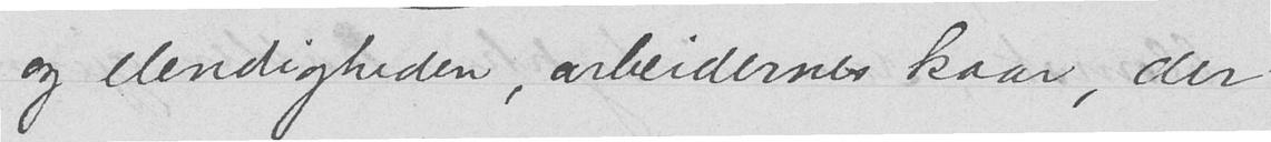og elendigheden, arbeidernes kaar, der
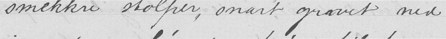smekkre stolper, snart gravet ned
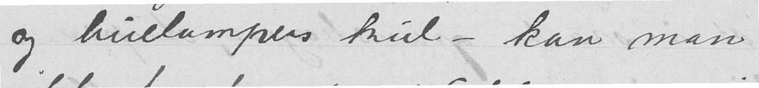og buelampers kul - kan man
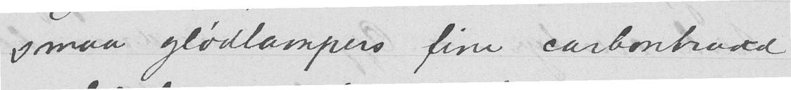smaa glødlampers fine carbontraad
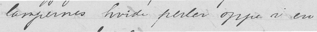lampernes hvide perler oppe i en
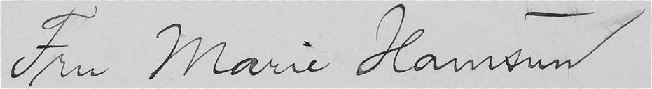Fru Marie Hamsun
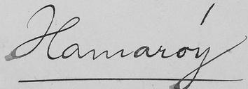Hamarøy
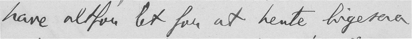have altfor let for at hente ligesaa
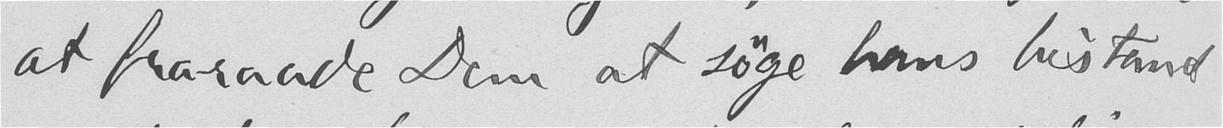at fraraade Dem at søge hans bistand
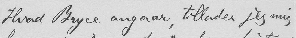Hvad Bryce angaar, tillader jeg mig
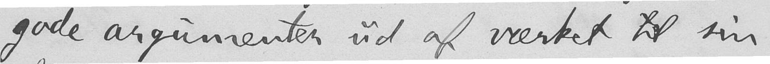gode argumenter ud af værket til sin
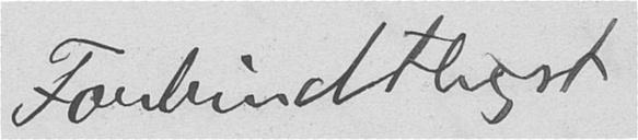Forbindtligst
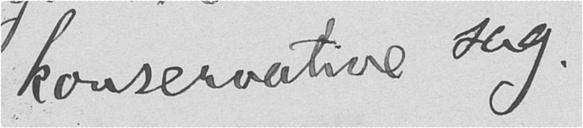konservative sag.
